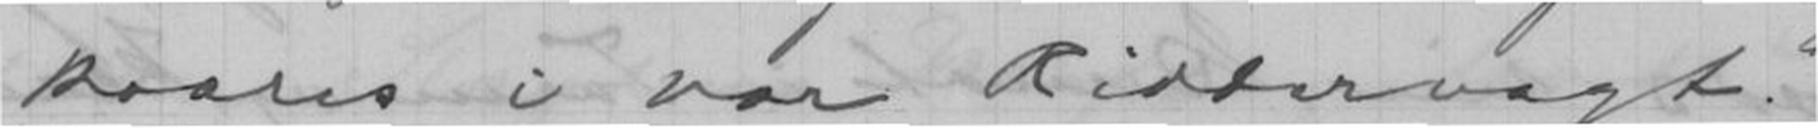kaares i vor Riddervagt"
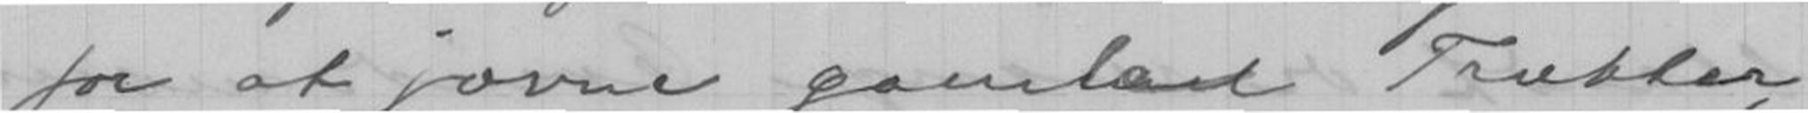for at jævne gamle Trætter,
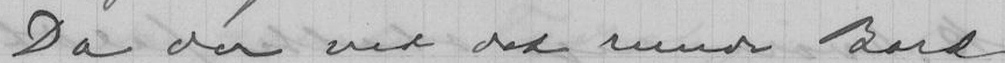Da der ved det runde Bord
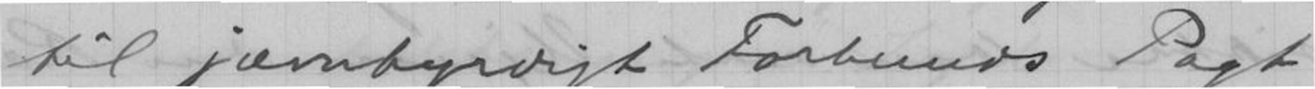til jævnbyrdigt Forbunds Pagt
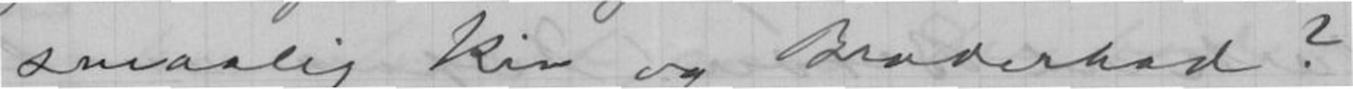Smaalig Kiv og Broderhad?
